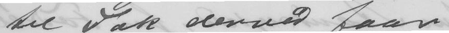til Tak derved faar
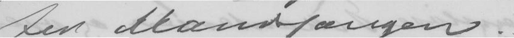for Mandsangen."
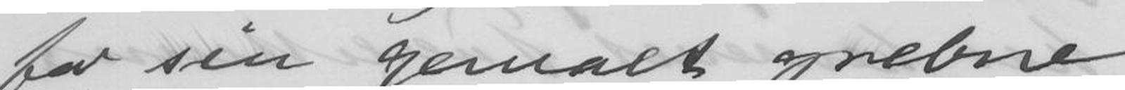for sin genialt grebne
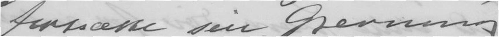forsætte sin Gjerning
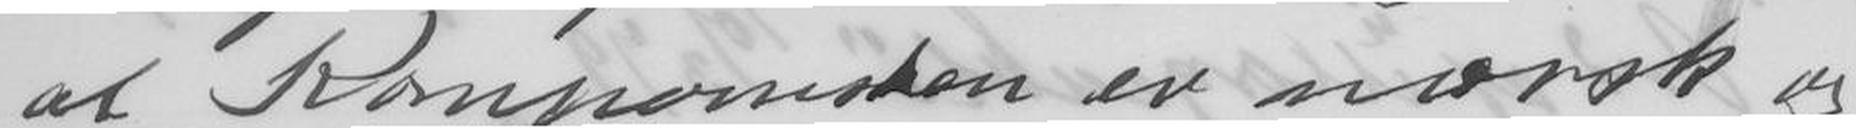at Komponisten er norsk og
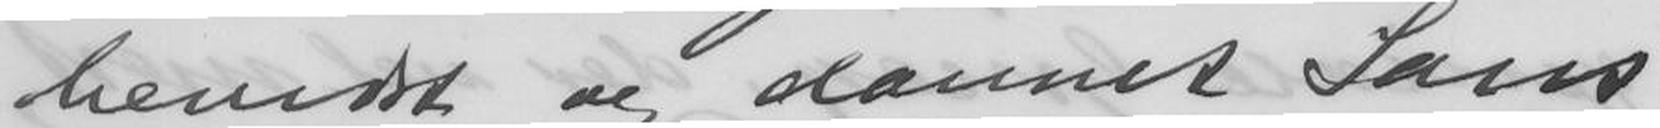bevidst og dannet Sans
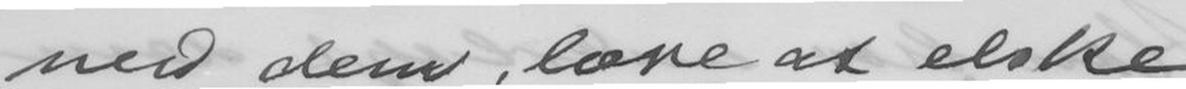med dem, lære at elske
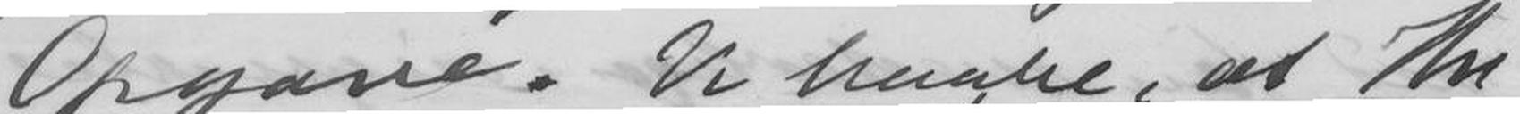Opgave. Vi haabe, at Hr
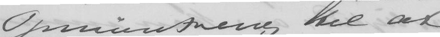Opmuntring til at
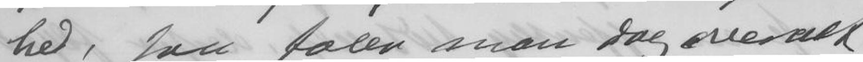hed, saa føler man dog overalt
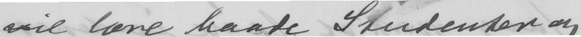vil lære baade Studenter og
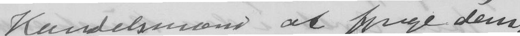Handelsmænd at synge dem
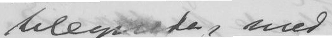tilegnede, med
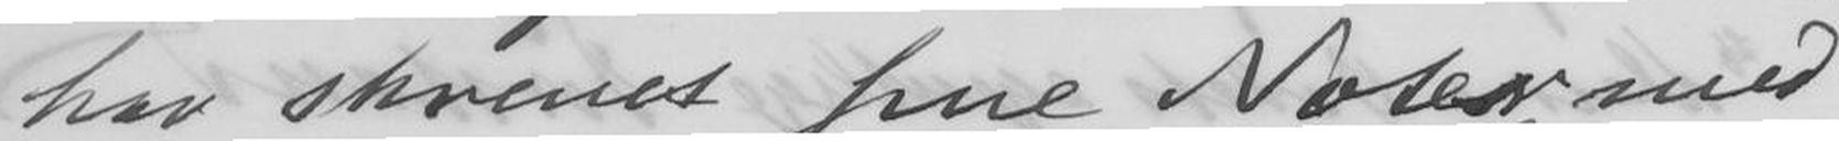har skrevet sine Noter med
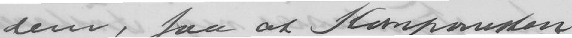dem, saa at Komponisten
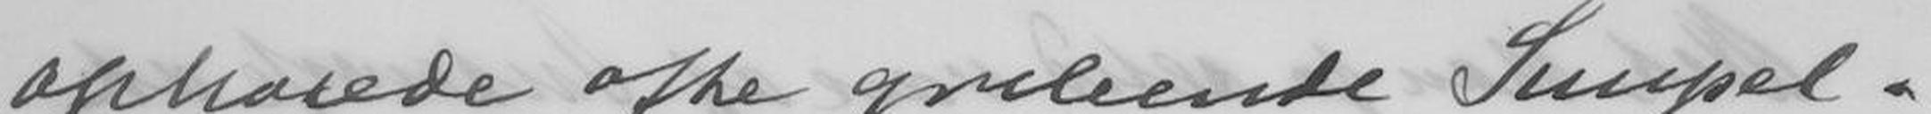ophavde ofte gribende Simpel-
